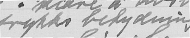trykks betydning!
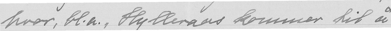hvor, bl.a., Hylleraas kommer til å
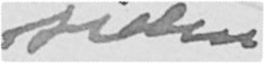siden
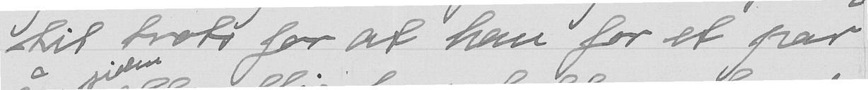til trots for at han for et par
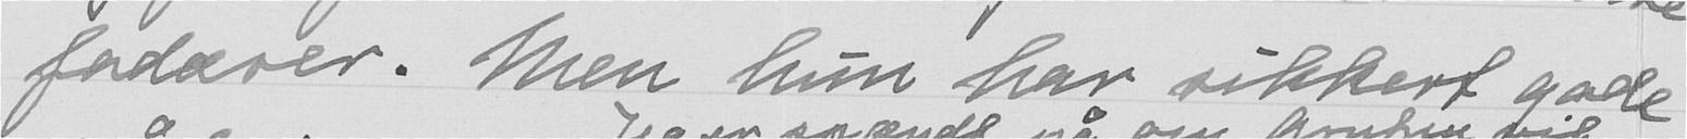fadæser. Men hun har sikkert gode
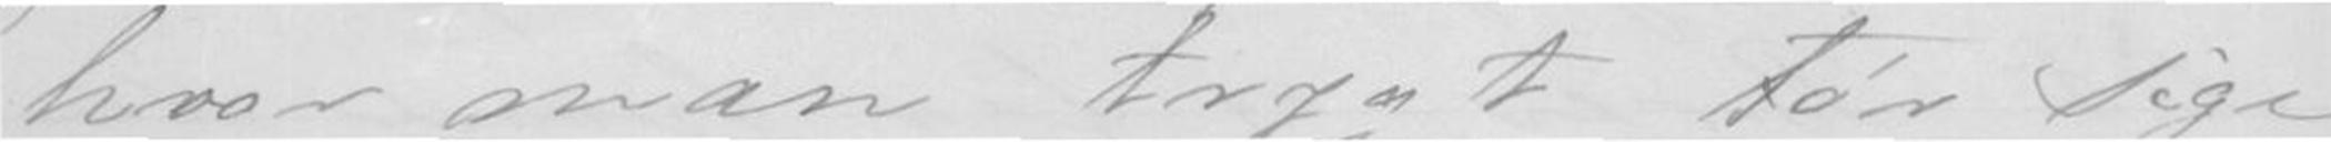hvor man trygt tør sige
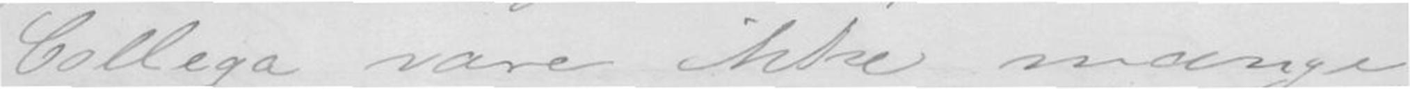Collega vare ikke mange,
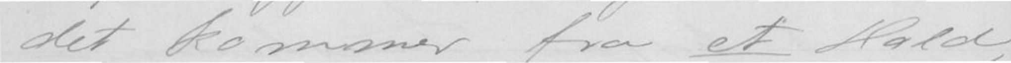det kommer fra et Hald,
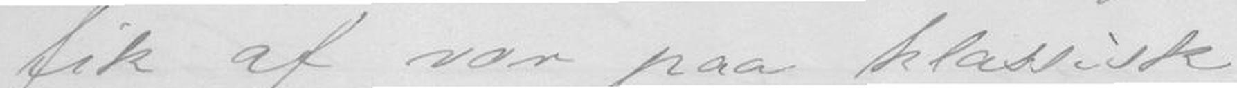fik af vor paa klassisk
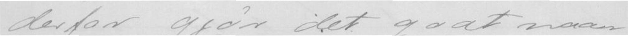derfor gjør det godt naar
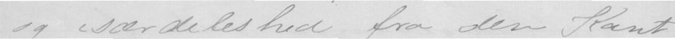og isærdeleshed fra den Kant
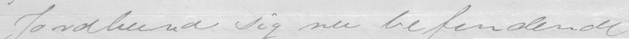Jordbund sig nu befindende
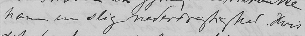ham en slig nederdrægtighed. Hvis
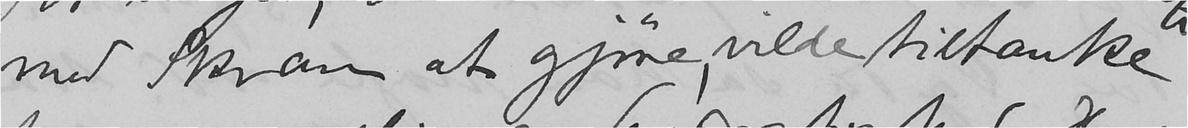med Skram at gjøre, vilde tiltænke
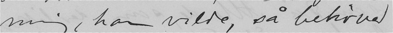mig, har vilde, så behøved
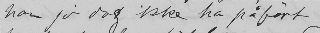han jo dog ikke ha påført
